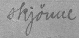skjønne
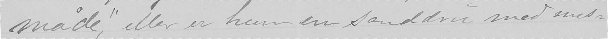måde", eller er hun en sanddru med mes-
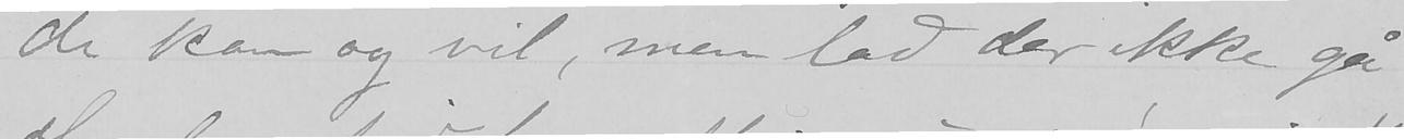de kan og vil, men lad der ikke gå
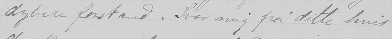dybere forstand. Svar mig på dette hvis
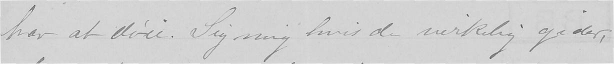har at døie. Sig mig hvis de virkelig gider,
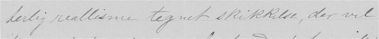terlig reallisme tegnet skikkelse, der vel
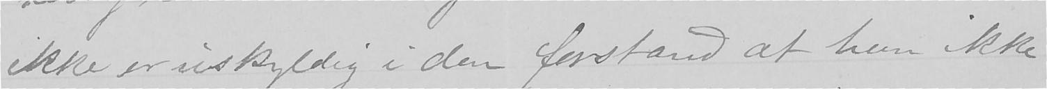ikke er uskyldig i den forstand at hun ikke
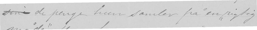sine de penge hun samler på en "rigtig
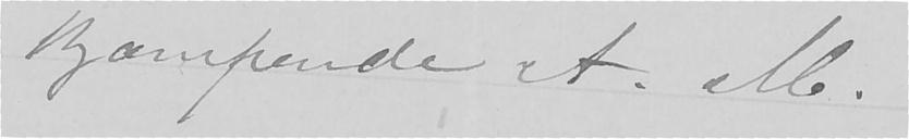kjæmpende A.M.
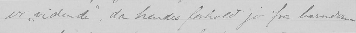er "vidende", da hendes forhold jo fra barndom
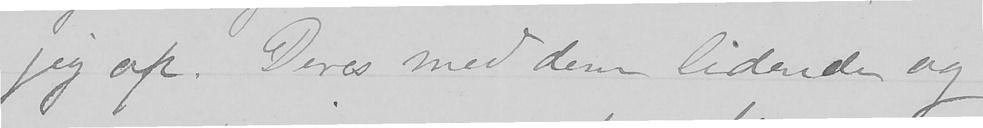jeg op. Deres med dem lidende og
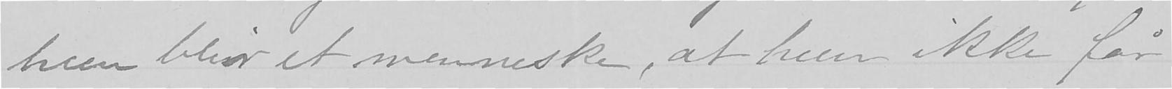hun blir et menneske, at hun ikke får
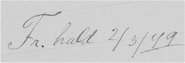Fr.hald 2/3/79.
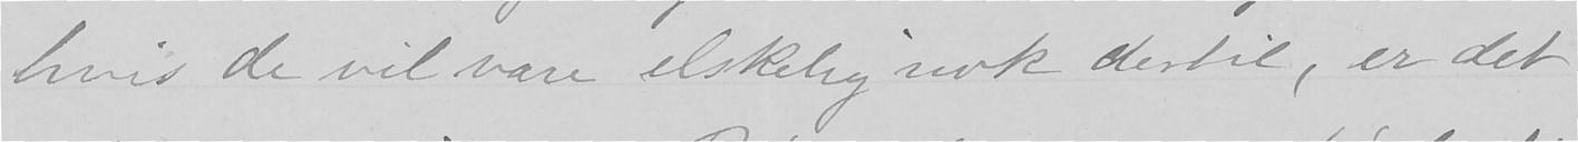hvis de vil være elskelig nok dertil, er det
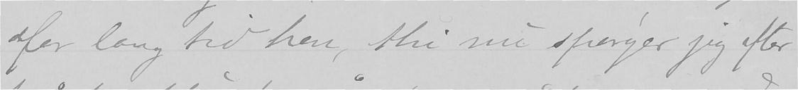for lang tid hen, thi nu spørger jeg efter
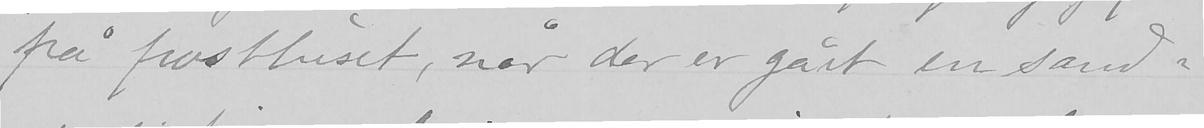på posthuset, når der er gået en sand-
